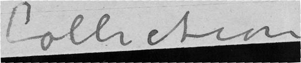Collection
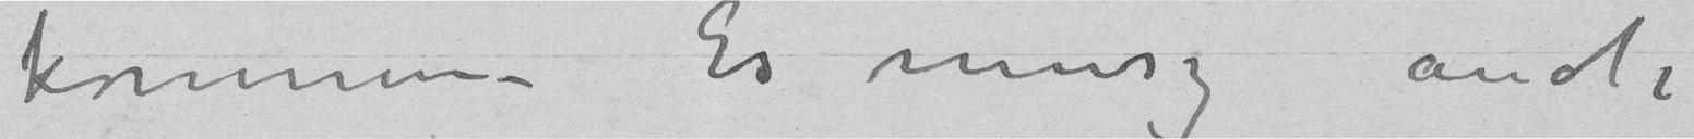kommen – Es musz auch
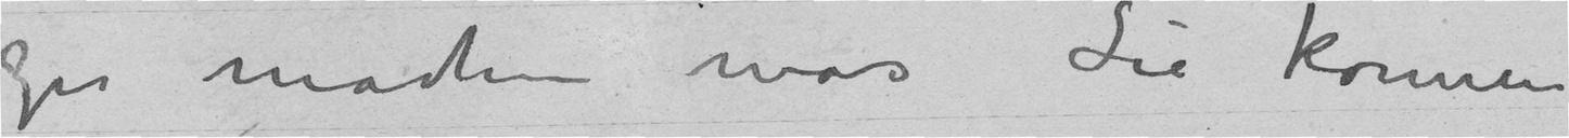zu machen was Sie konnen
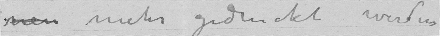neu mehr gedruckt werden
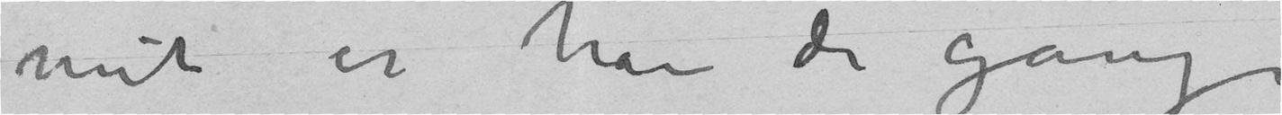mit er hat die ganze
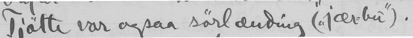Tjøttø var ogsaa sørlænding ("jær-bu").
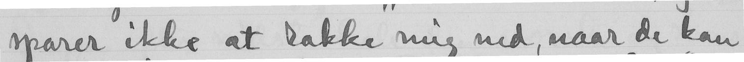sparer ikke at rakke mig ned, naar de kan
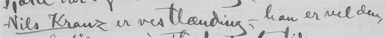Nils Kranz er vestlænding,- han er vel den,
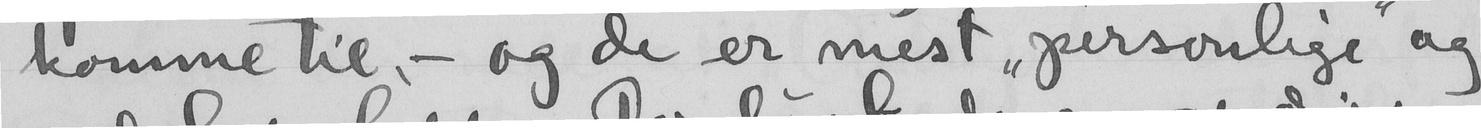komme til, - og de er mest "personlige" og
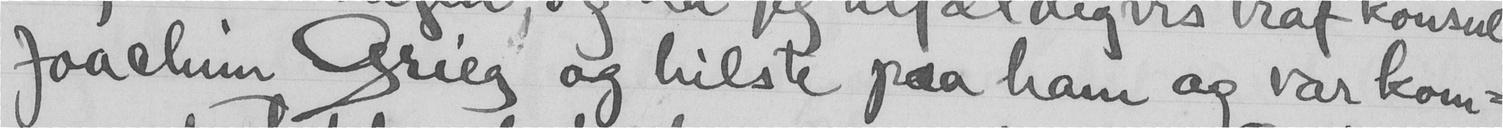Joachim Grieg og hilste paa ham og var kom-
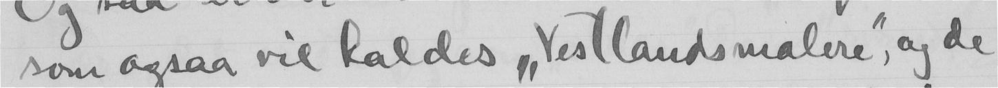som ogsaa vil kaldes "Vestlandsmalere", og de
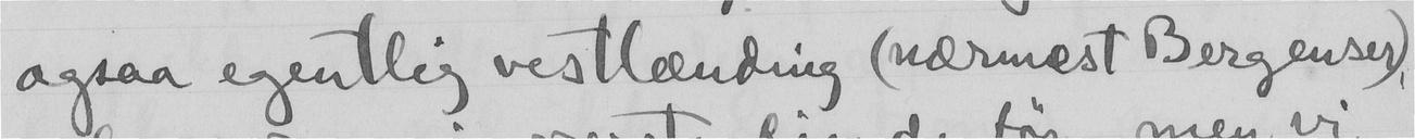ogsaa egentlig vestlænding (nærmest Bergenser)
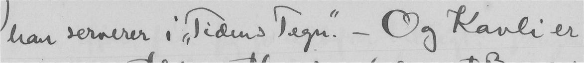han serverer i "Tidens Tegn". - Og Kavli er
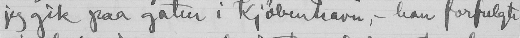jeg gik paa gaten i Kjøbenhavn, - han forfulgte
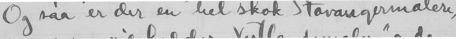Og saa er der en hel skok stavangermalere,
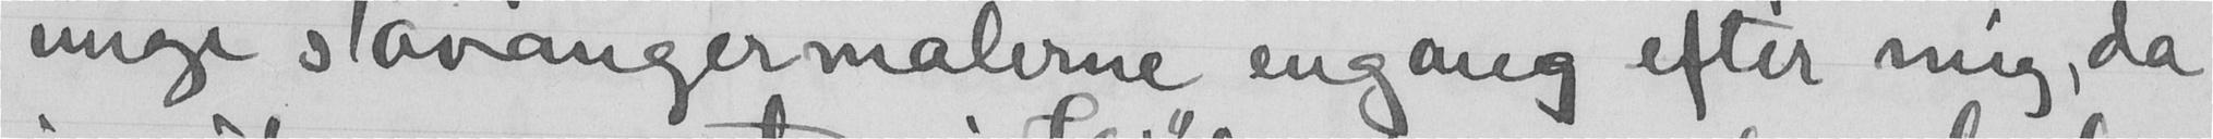unge stavangermalerne engang efter mig, da
